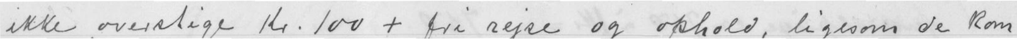ikke overstige Kr. 100 + fri rejse og ophold, ligesom de Kom
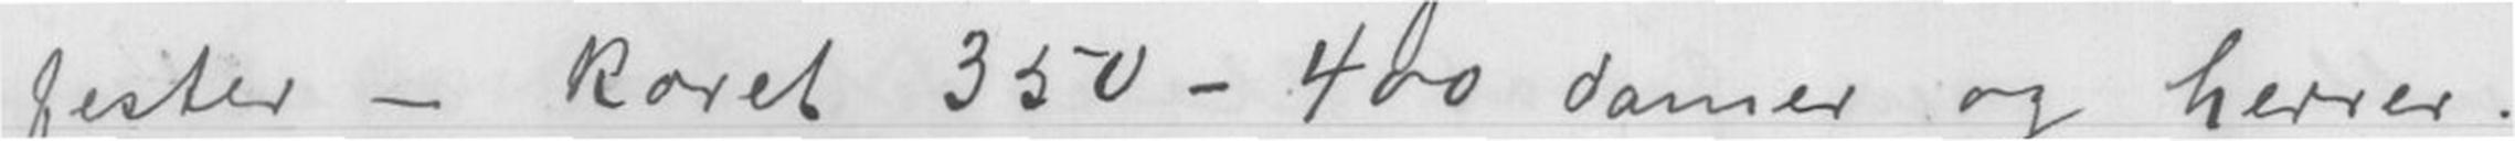fester - Koret 350 - 400 damer og herrer.
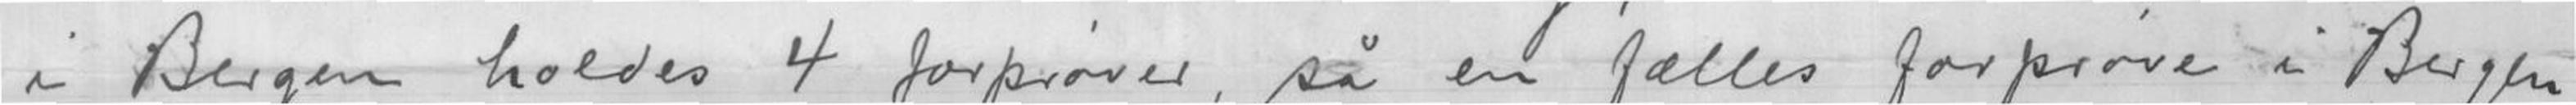i Bergen holdes 4 forprøver, så en fælles forprøve i Bergen
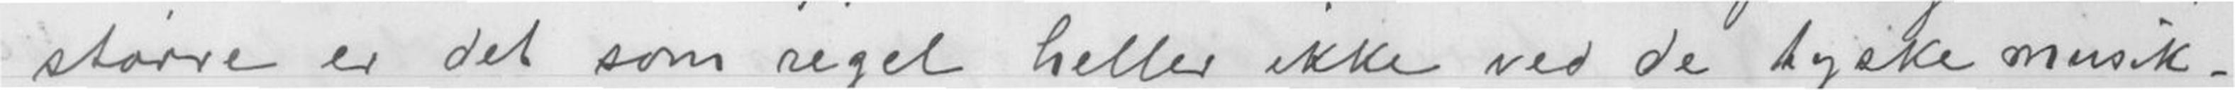- større er det som regel heller ikke ved de tyske musik-
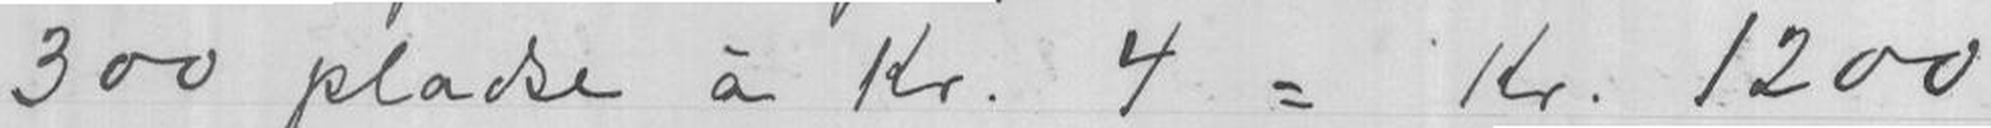300 pladse à Kr. 4 = Kr. 1200
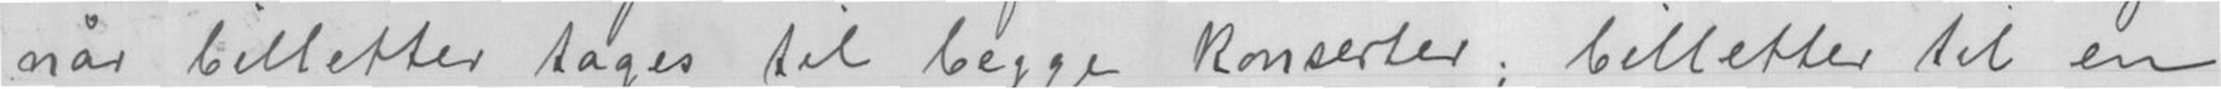når billetter tages til begge Konserter; billetter til en
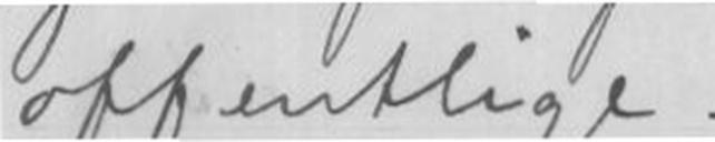offentlige.
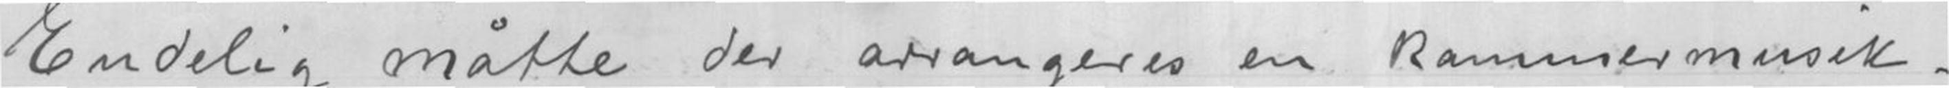Endelig måtte der arrangeres en Kammermusik-
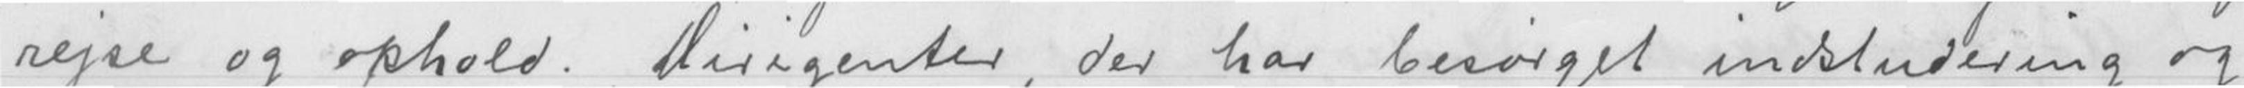rejse og ophold. Dirigenter, der har besørget indstudering og
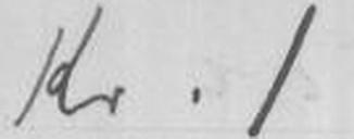Kr.1.
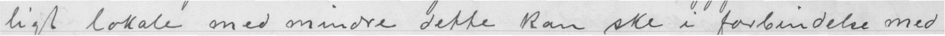ligt lokale med mindre dette kan ske i forbindese med
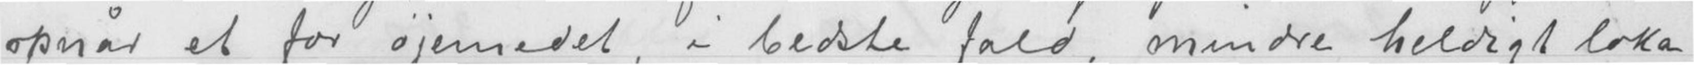opnår et for øjemedet, i bedste fald, mindre heldigt loka-
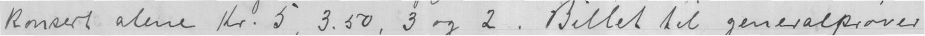Konsert alene Kr. 5, 3.50, 3 og 2. Billet til generalprøver
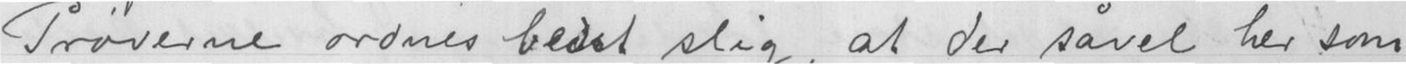Prøverne ordnes bedst slig, at der såvel her som
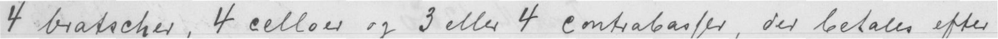4 bratscher, 4 celloer og 3 eller 4 contrabasser, det betales efter
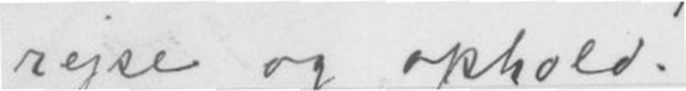rejse og ophold.
